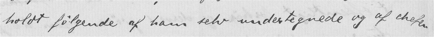holdt følgende af ham selv undertegnede og af chefen
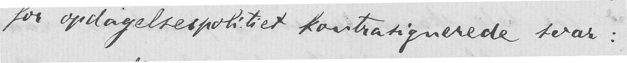for opdagelsespolitiet kontrasignerede svar:
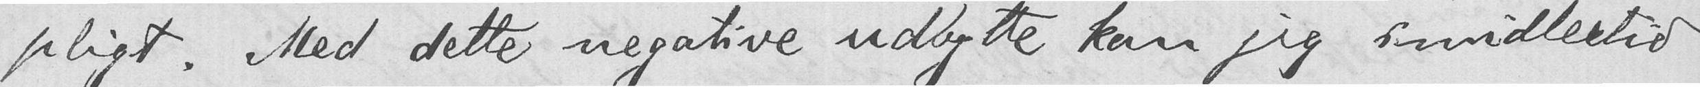pligt. Med dette negative udbytte kan jeg imidlertid
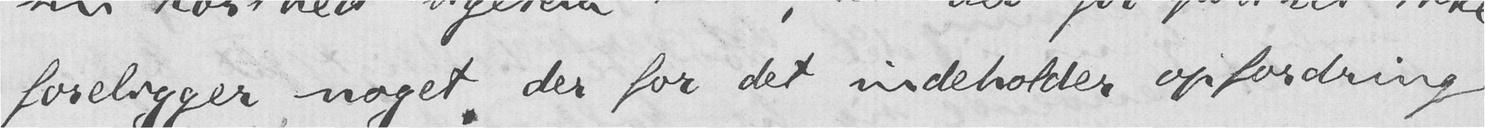foreligger noget, der for det indeholder opfordring
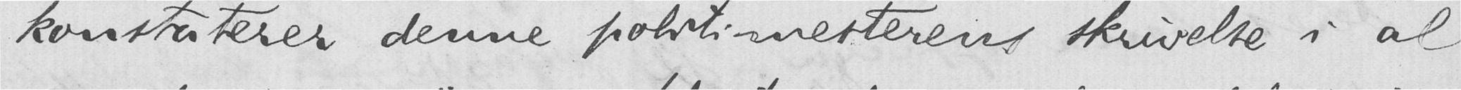konstaterer denne politimesterens skrivelse i al
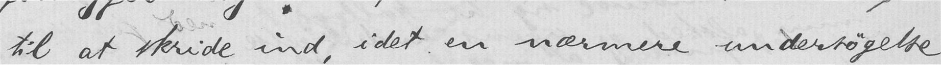til at skride ind, idet en nærmere undersøgelse
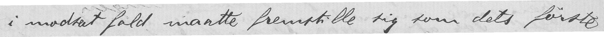i modsat fald maatte fremstille sig som dets første
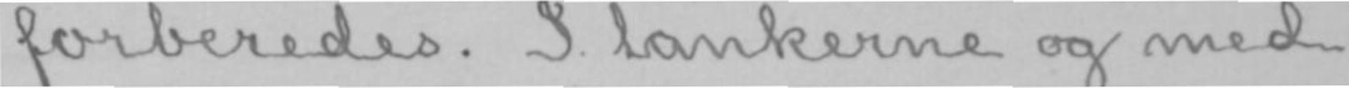forberedes. I tankerne og med
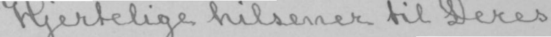Hjertelige hilsener til Deres
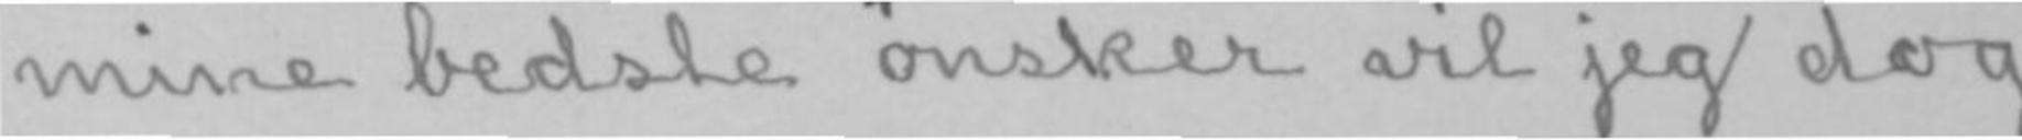mine bedste ønsker vil jeg dog
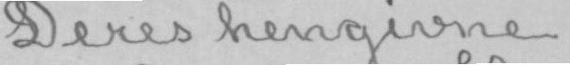Deres hengivne
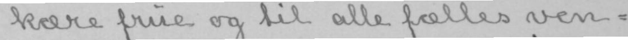kære frue og til alle fælles ven-
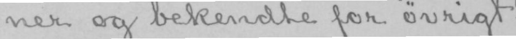ner og bekendte for øvrigt!
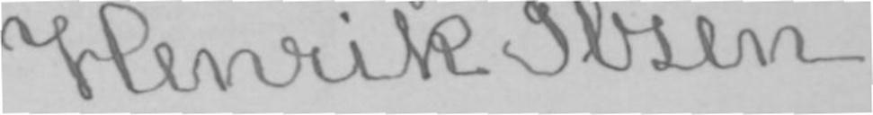Henrik Ibsen.
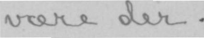være der.
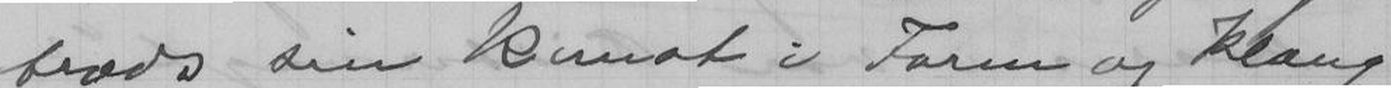trods sin Kunst i Form og Klang
